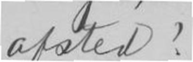afsted!
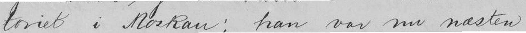toriet i Moskau; han var nu næsten
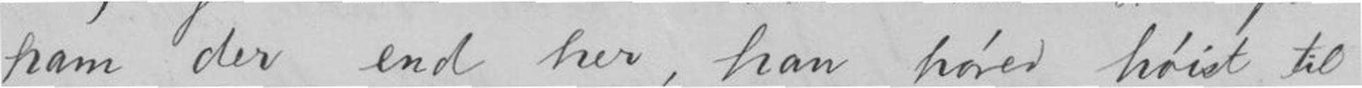ham der end her, han hører høist til
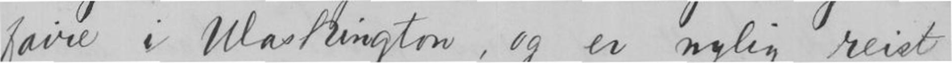faire i Washington, og er nylig reist
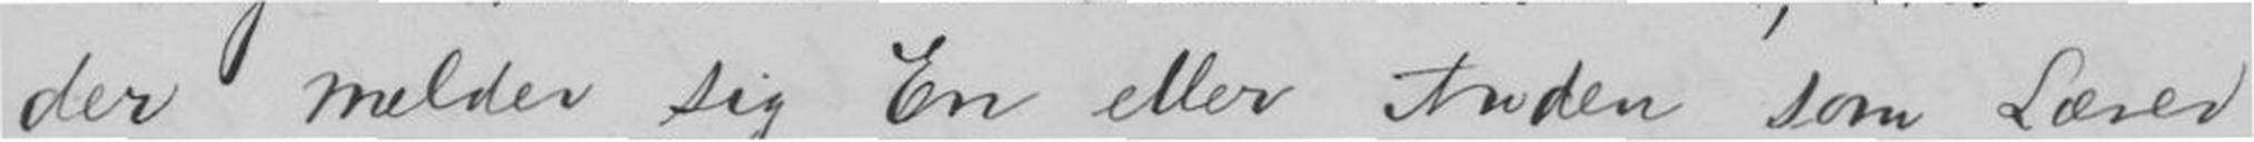der melder sig En eller Andern som Lærer
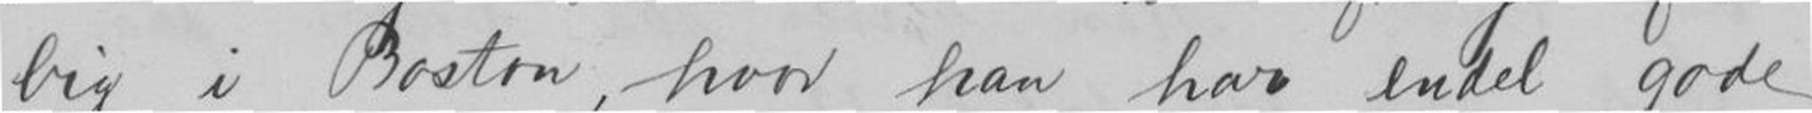big i Boston, hvor han har endel gode
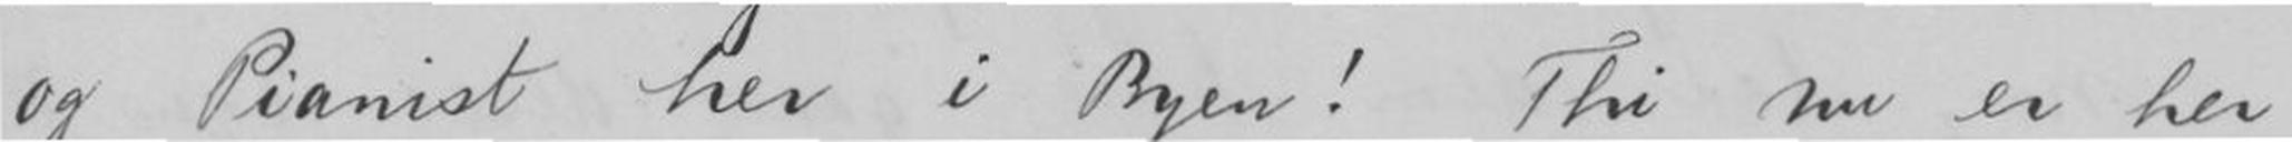og Pianist her i Byen! Thi nu er her
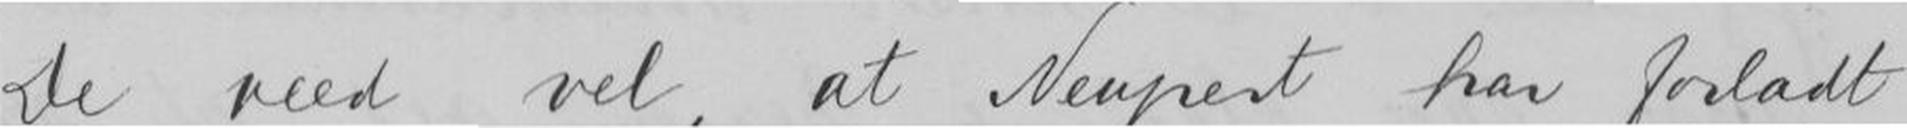De veed vel at Neupert har forladt
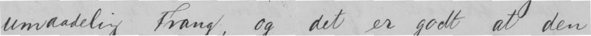umaadelig Trang, og det er godt at den
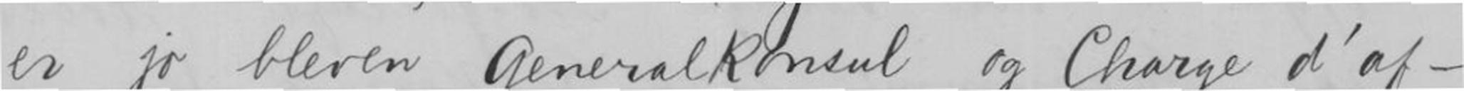er jo bleven Generalkonsul og Charge d'af-
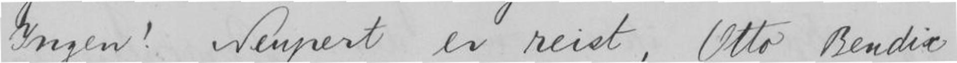Ingen! Neupert er reist, Otto Bendix
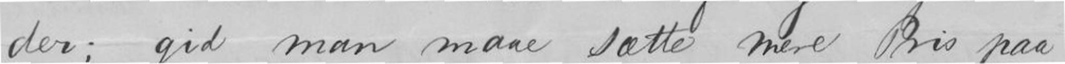der; gid man maae sætte mere Pris paa
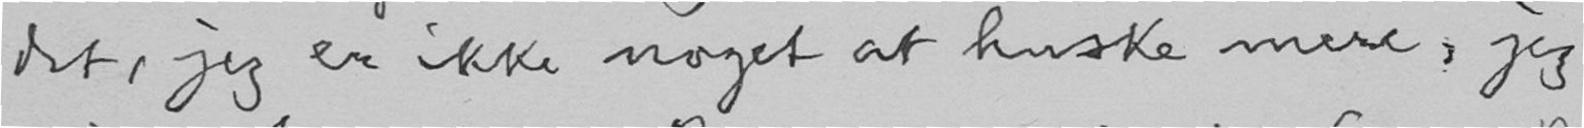det, jeg er ikke noget at huske mere, jeg
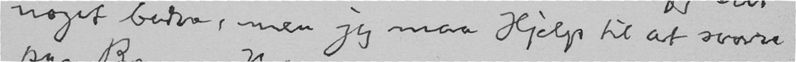noget bedre, men jeg maa Hjelp til at svare
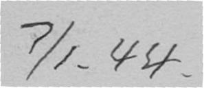7/1. 44.
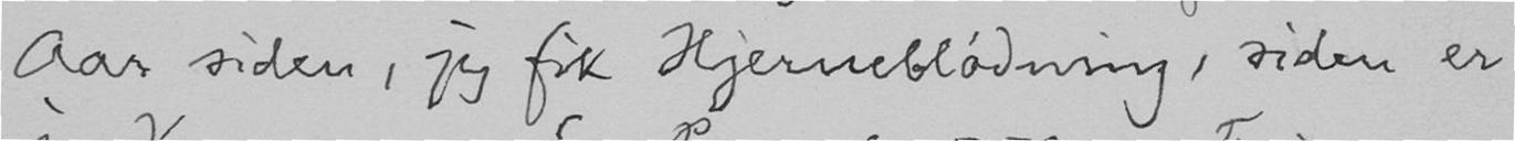Aar siden, jeg fik Hjerneblødning, siden er
